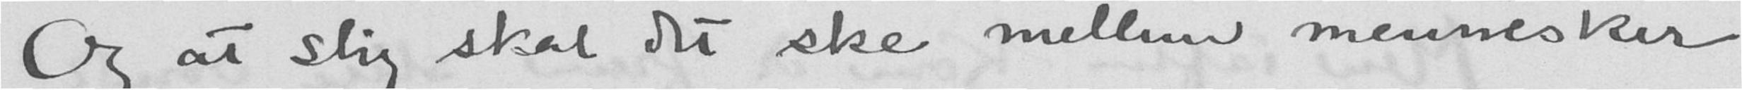Og at slig skal det ske mellem mennesker
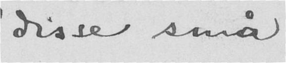disse små
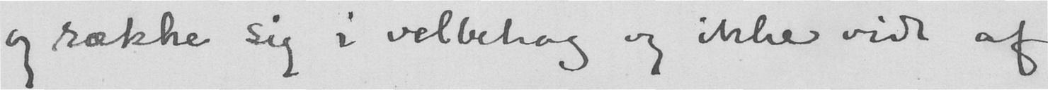og række sig i velbehag og ikke vide af
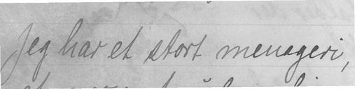Jeg har et stort menageri,
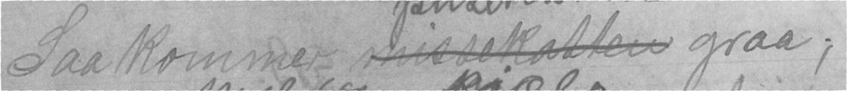Saa kommer pusekatten graa;
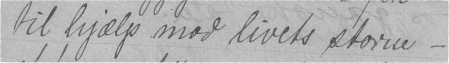til hjælp mod livets storm -
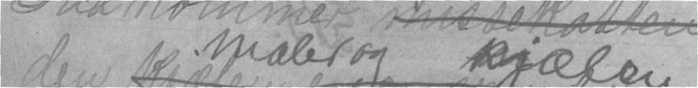den maler og kjælen
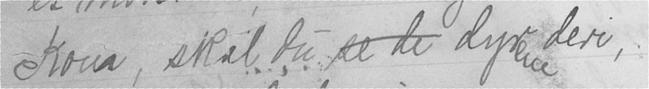Kom, skal du se de dyrene deri,
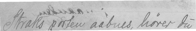Straks porten aabnes, hører du
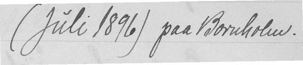(Juli 1896) paa Bornholm.
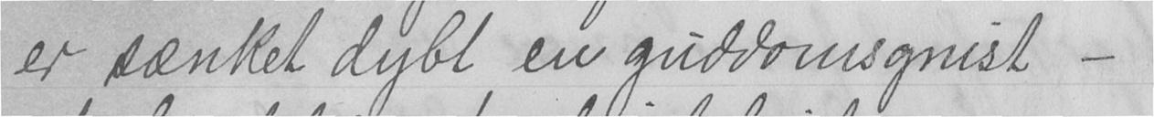er sænket dybt en guddomsgnist -
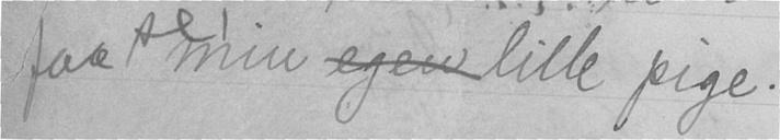faa se min egen lille pige.
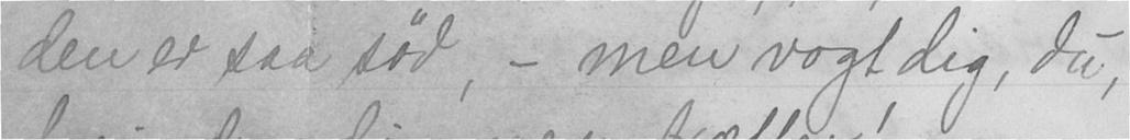den er saa sød, - men vogt dig, du,
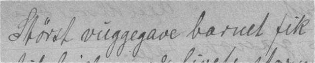Størst vuggegave barnet fik
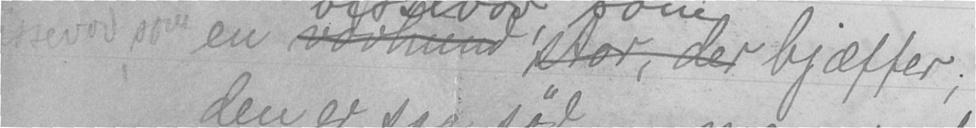en bissevov som bjæffer;
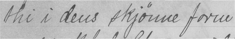thi i dens skjønne form
